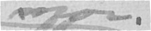mor.
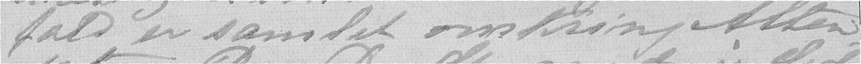fald er samlet omkring Alter-
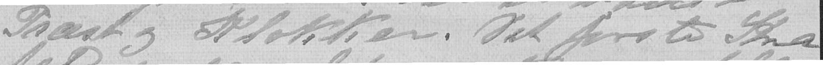Præst og Klokker. Det første Knæ-
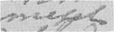meget
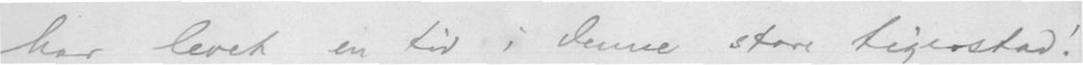har levet en tid i denne store tigerstad!
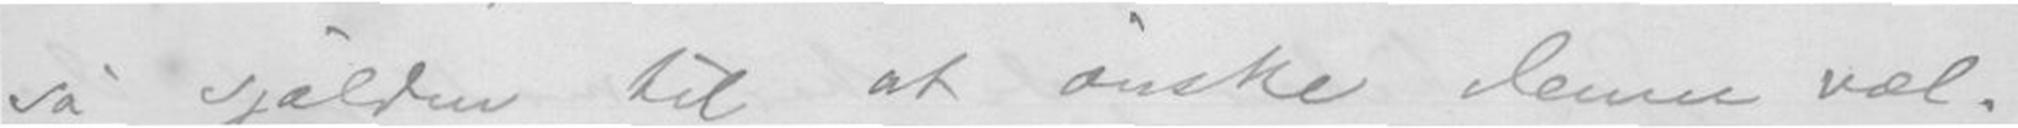så sjælden til at ønske denne væl-
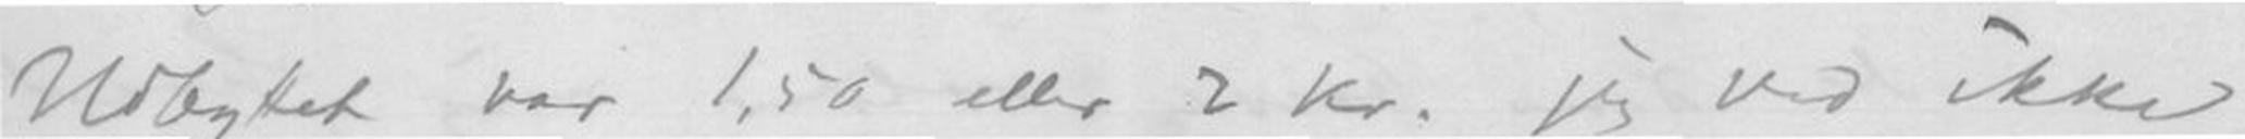Udbyttet var 1,50 eller 2 kr. jeg ved ikke
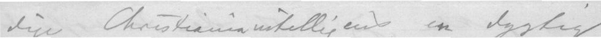dige Christianiaustillings en dygtig
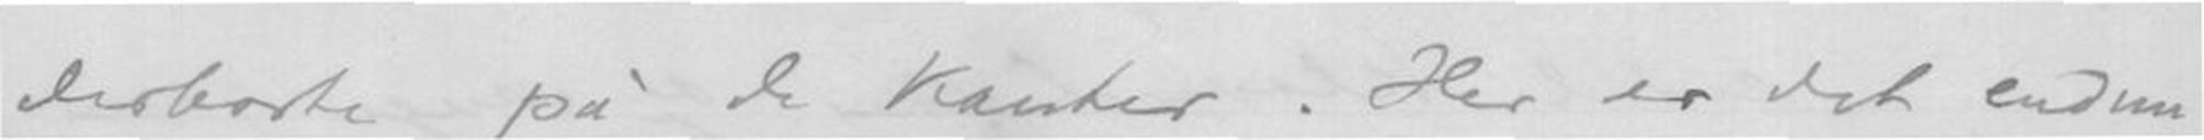derborte på de kanter. Her er det endnu
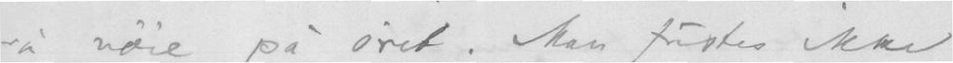så nøie på øret. Man fristes ikke
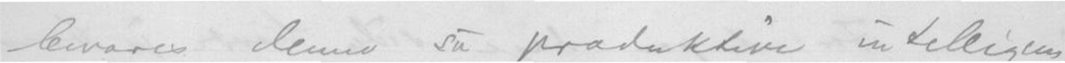bevares denne så produktive intelligens
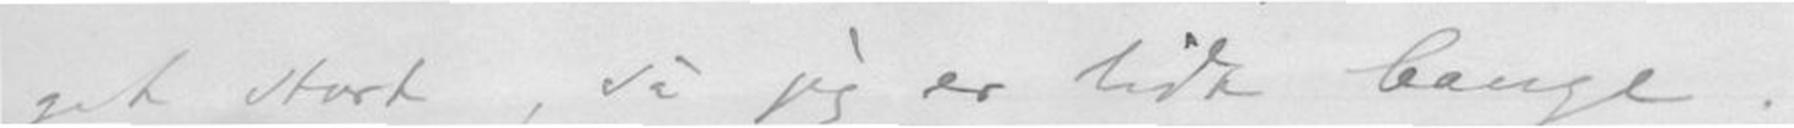get stort, så jeg er lidt bange.
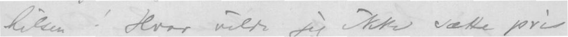hilsen! Hvor vilde jeg ikke sætte pris
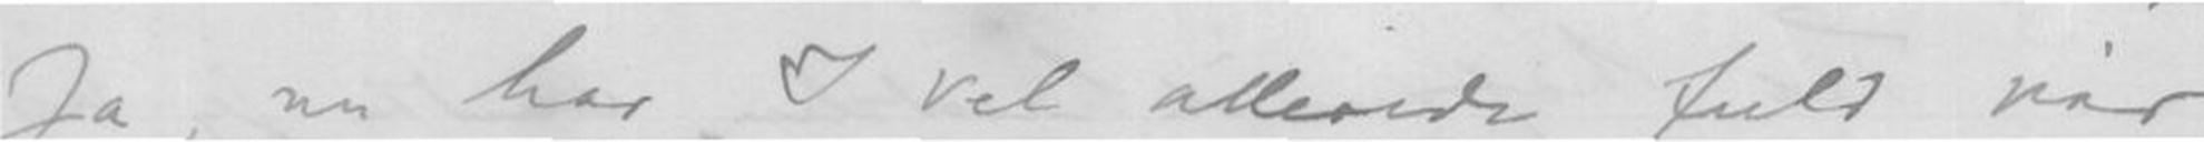Ja, nu har I vel allerede fuld vår
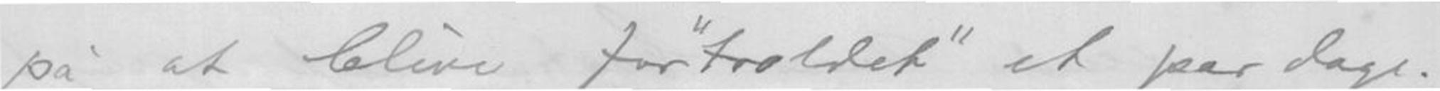på at blive for "troldet"et par dage.
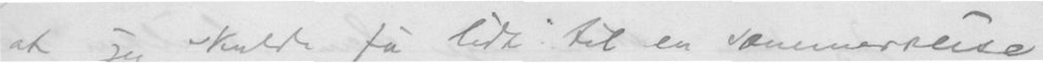at jeg skulde få lidt til en sommerreise.
